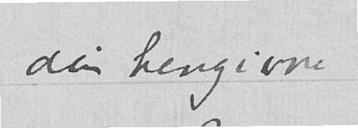din hengivne
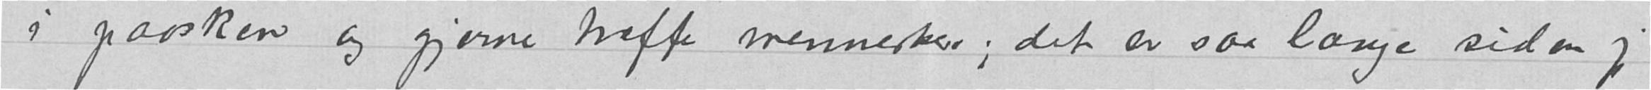i paasken og gjerne træffe mennesker; det er saa længe siden jeg
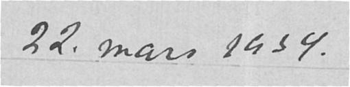22. mars 1934.
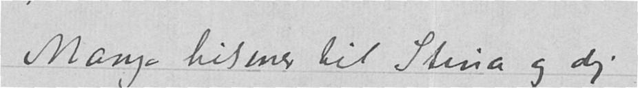Mange hilsener til Stina og dig
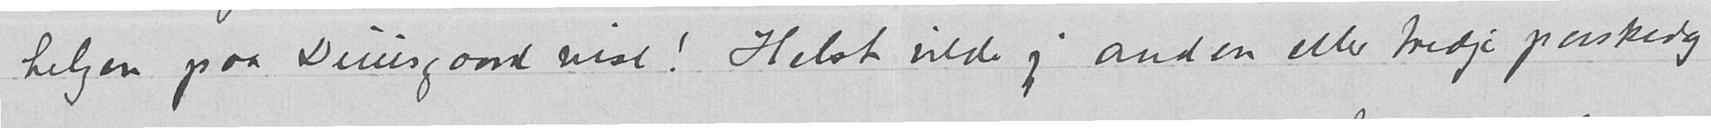helgen paa Duusgaard vist! Helst vilde jeg anden eller tredje paaskedag
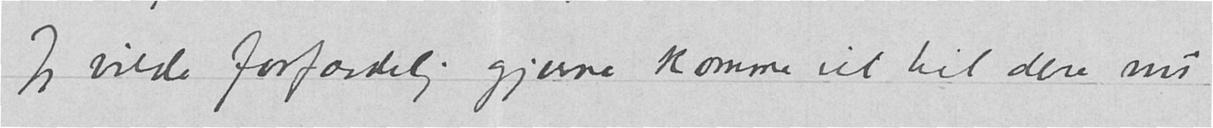Jeg vilde forfærdelig gjerne komme ut til dere nu
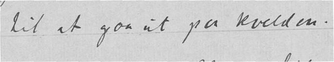til at gaa ut paa kvelden.
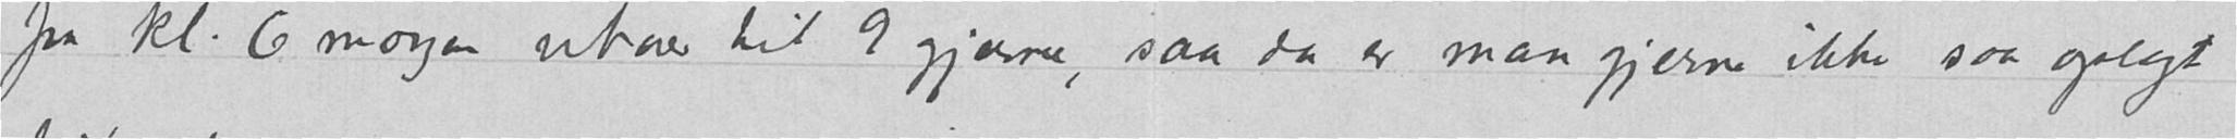fra kl. 6 morgen utover til 9 gjerne, saa da er man gjerne ikke saa oplagt
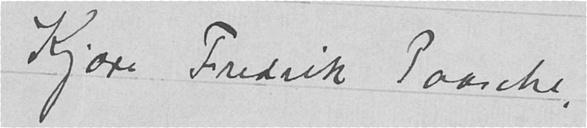Kjære Fredsik Paasche,
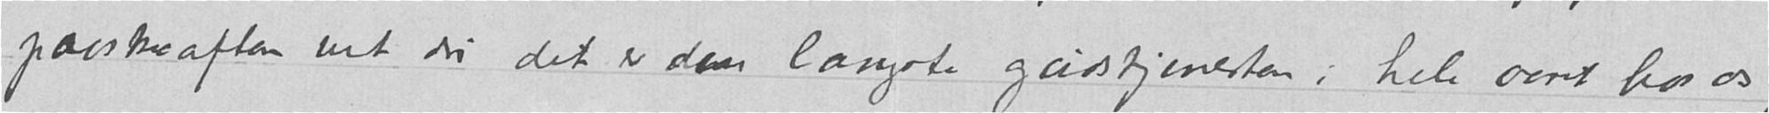- paaskeaften vet du det er den længste gudstjenesten i hele aaret hos os,
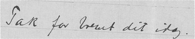Tak for brevet dit idag.
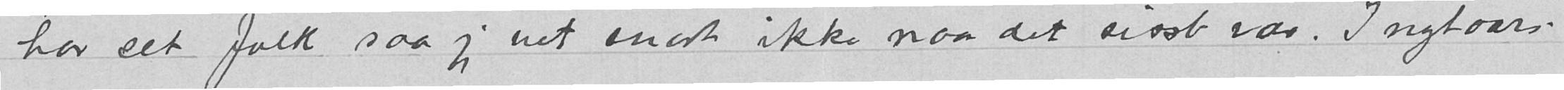har set folk saa jeg vet snart ikke naar det sisst var. I nytaars-
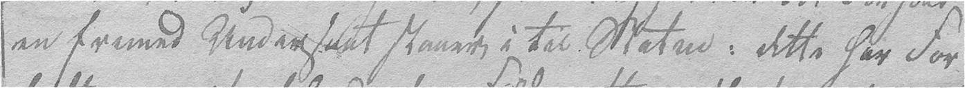en fremmed Undersaat staaer i til Staten: dette har For-
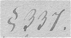§ 337.
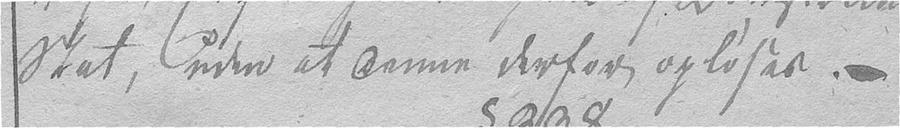Stat, uden at denne derfor opløses. –
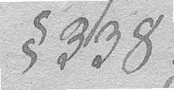§ 338.
